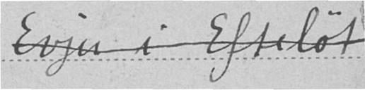Evju i Efteløt
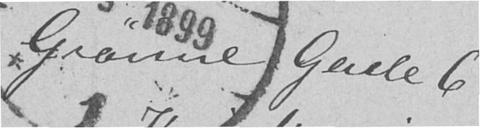Grønne Gade 6
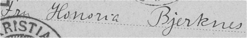Fru Honoria Bjerknes
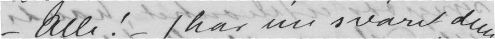- Alle! - J har nu svaret dem
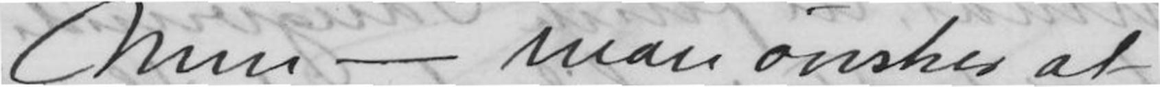Men - man ønsker at
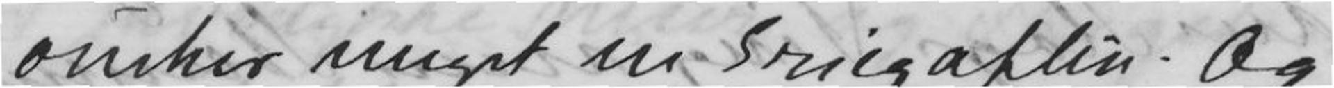ønsker meget en Griegaften. Og
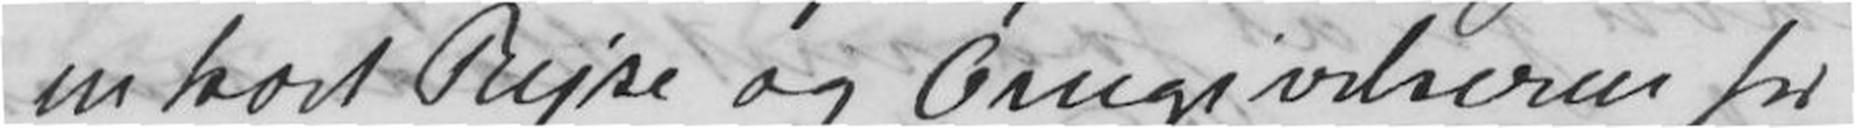en kort Rejse og Omgivelserne for
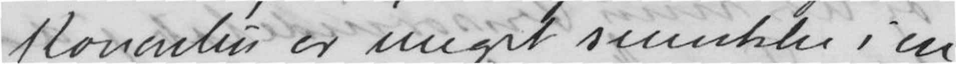Koncerten er meget smukke i en
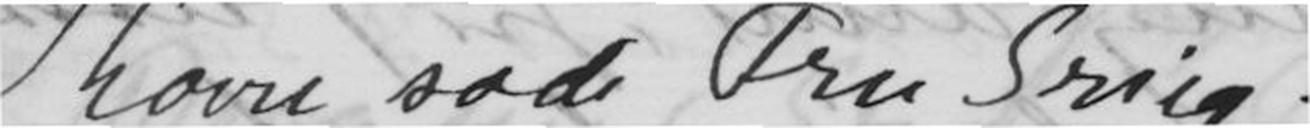Kaere søde Fru Grieg!
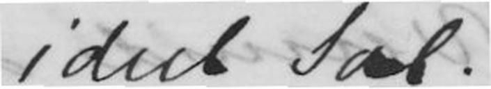ideel Sal.
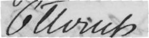Otterup
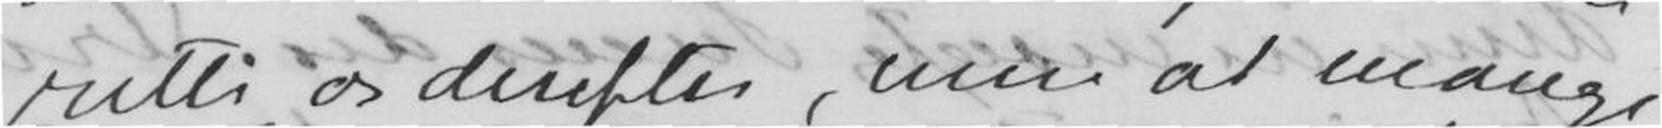rette os derefter, men at mange
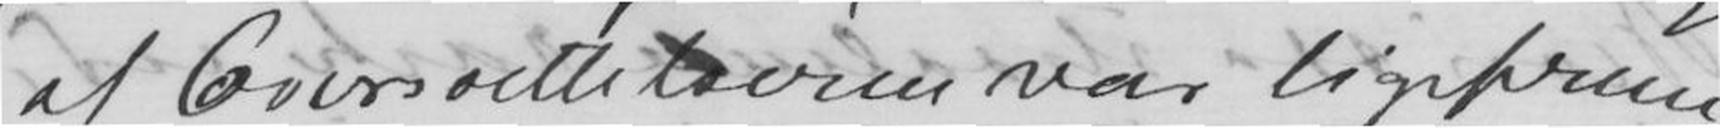af Oversaettelserne var ligefrem
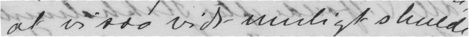at vi saa vidt muligt skulde
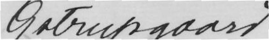Østrupgaard
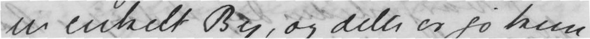en enkelt By, og dette er jo kun
